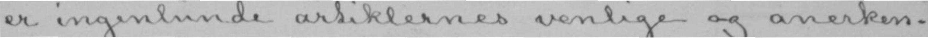er ingenlunde artiklernes venlige og anerken-
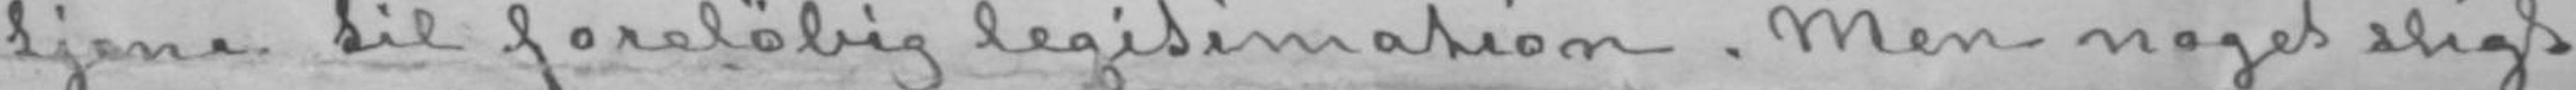tjene til foreløbig legitimation. Men noget sligt
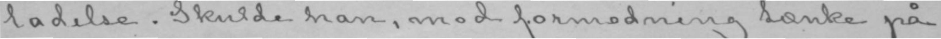ladelse. Skulde han, mod formodning tænke på
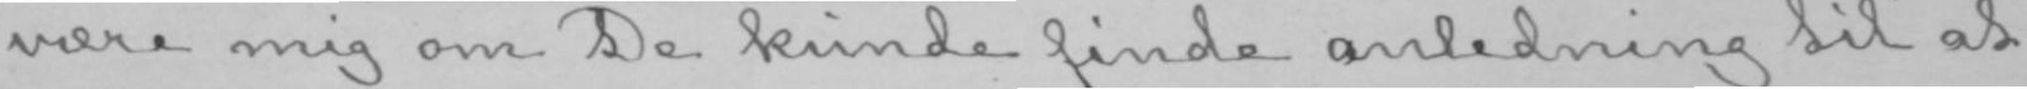være mig om De kunde finde anledning til at
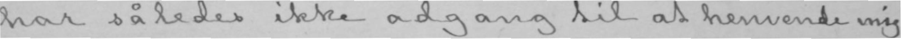har således ikke adgang til at henvende mig
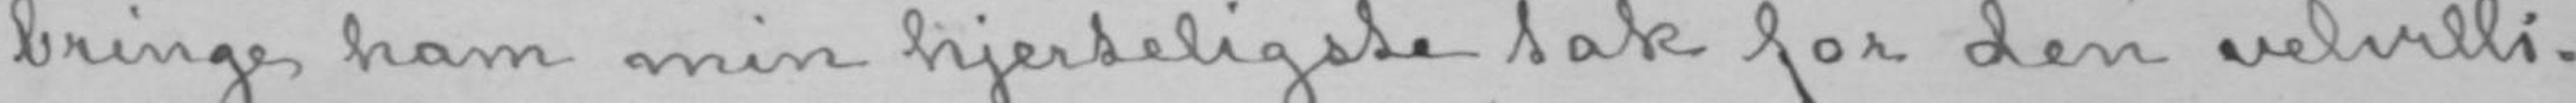bringe ham min hjerteligste tak for den velvilli-
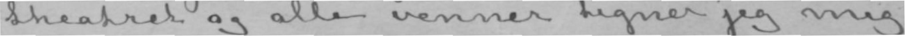theatret og alle venner tegner jeg mig
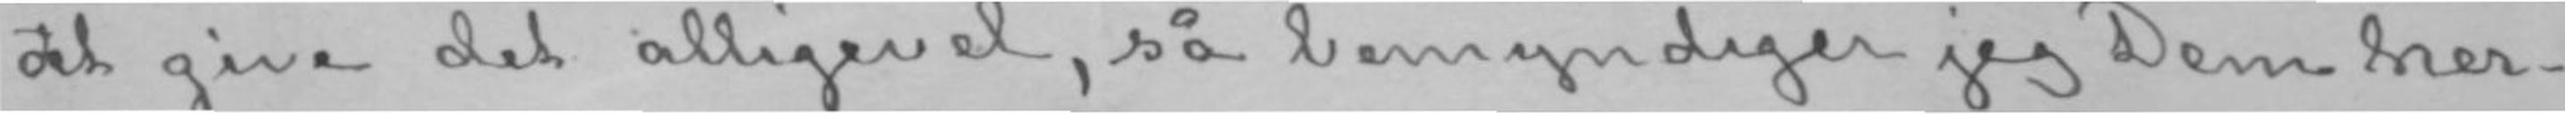dat give det alligevel, så bemyndiger jeg Dem her-
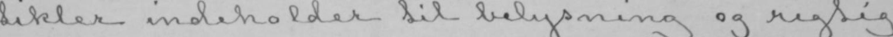tikler indeholder til belysning og rigtig
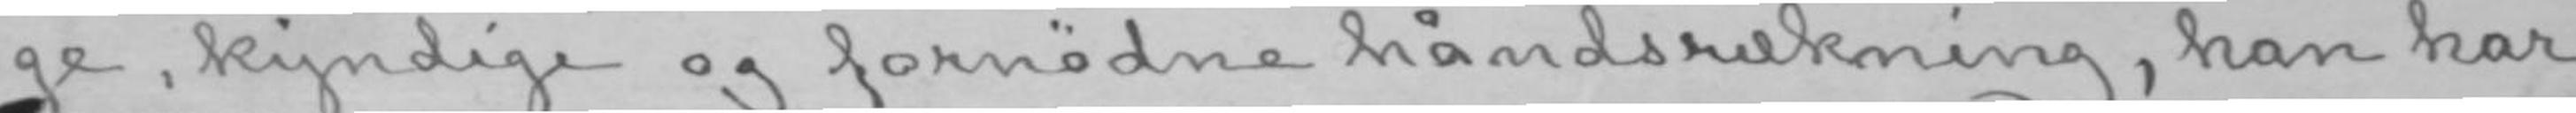ge, kyndige og fornødne håndsrækning, han har
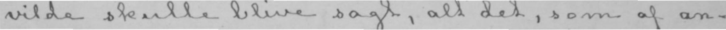vilde skulle blive sagt, alt det, som af an-
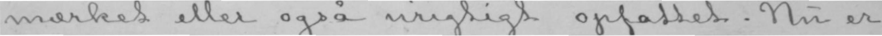mærket eller også urigtigt opfattet. Nu er
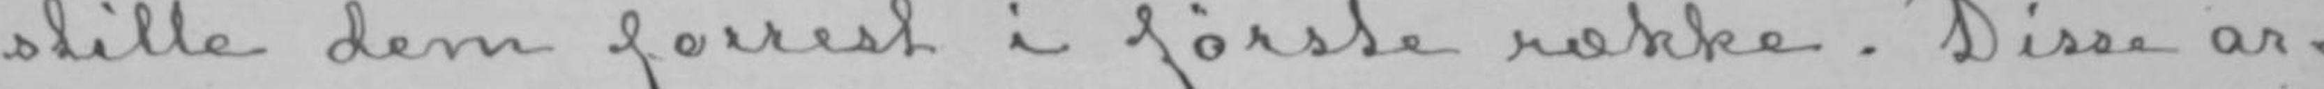stille dem forrest i første række. Disse ar-
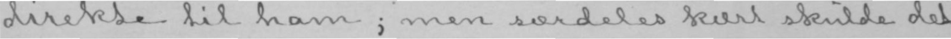direkte til ham; men særdeles kært skulde det
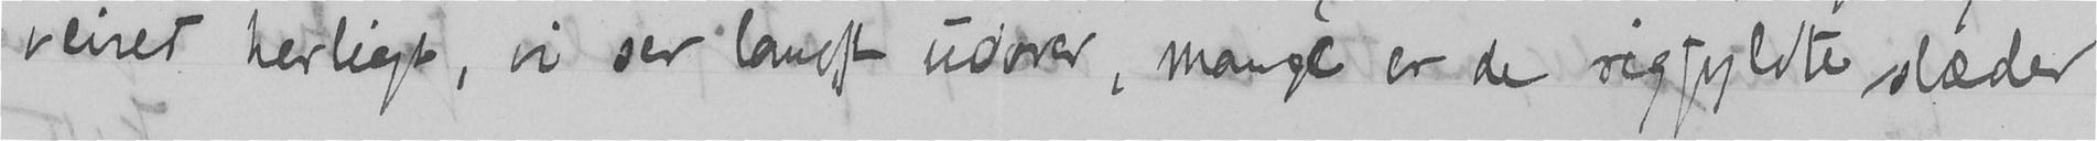veiret herligt, vi ser langt udover, mange er de rigfyldte slæder
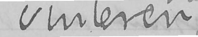vinteren
